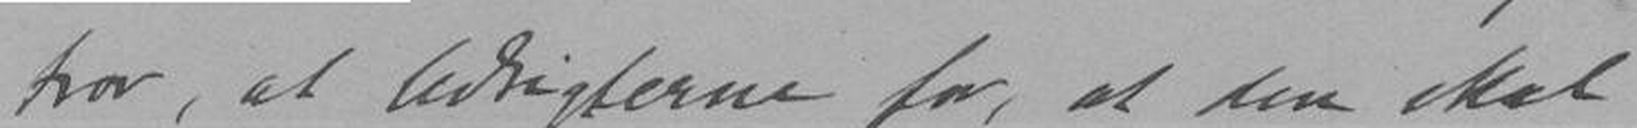tror, at Udsigterne for, at den skal
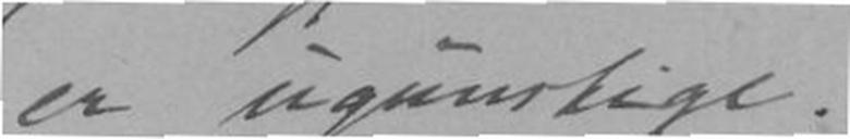er ugunstige.
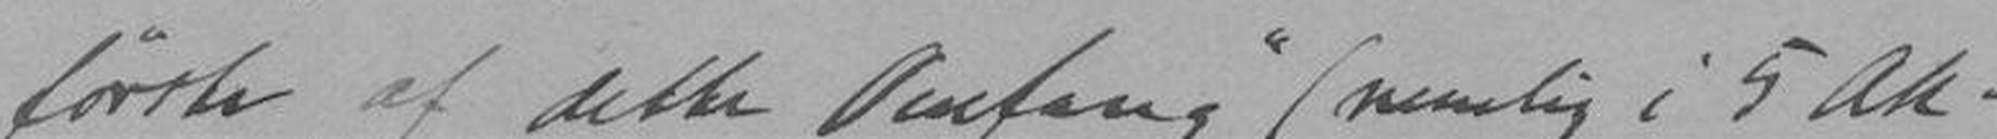første af dette Omfang" (nemlig i 5 Ak-
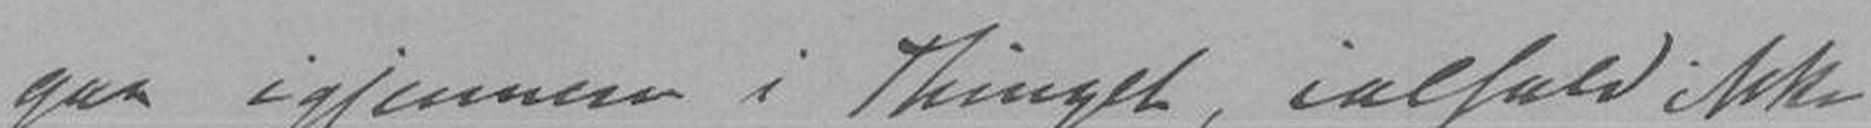gaa igjennem i Thinget, ialfald ikke
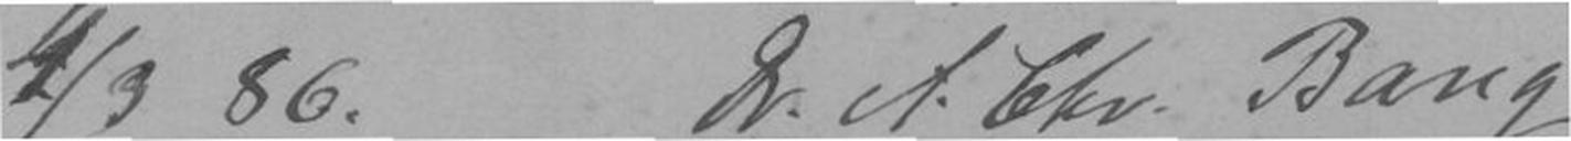4/3 86Dr. A. Chr Bang
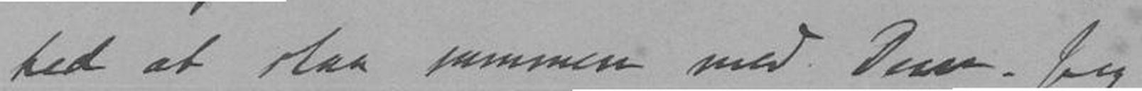hed at staa sammen med Dem. Jeg
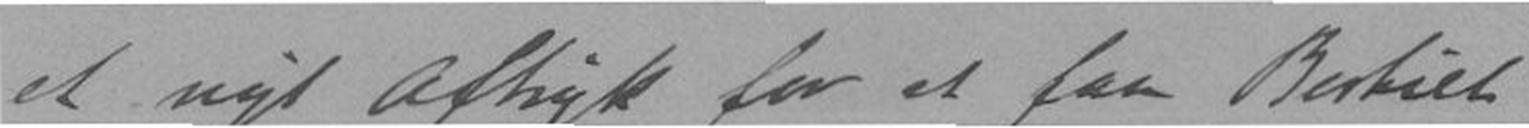et nyt Aftryk for et faa Bestilte
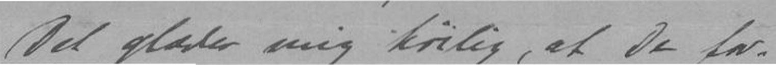Det glæder mig høilig, at De for-
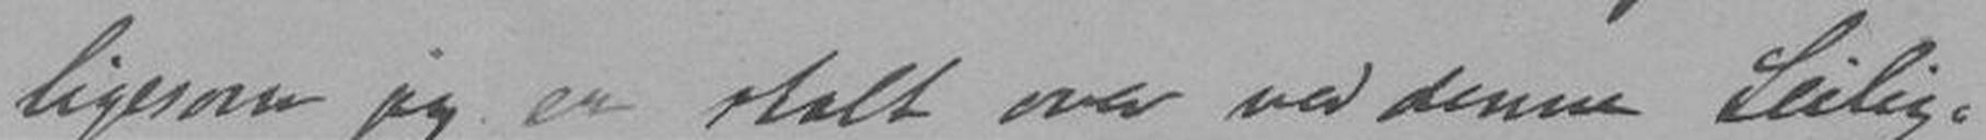ligesom jeg er stolt over ved denne Leilig-
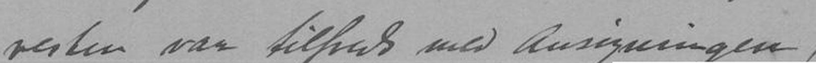resten var tilfreds med Ansøgningen,
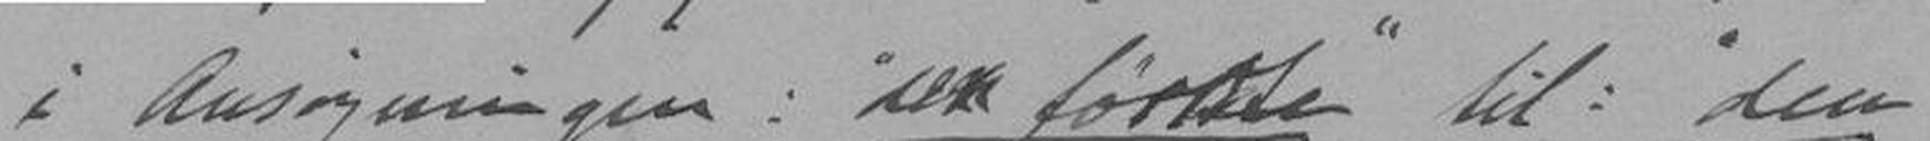i Ansøgningen: "den førdte" til: "den
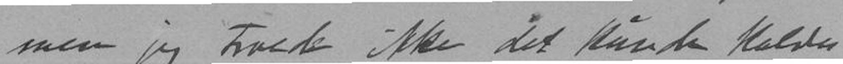men jeg Troede ikke det kunde kaldes
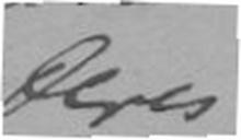Deres
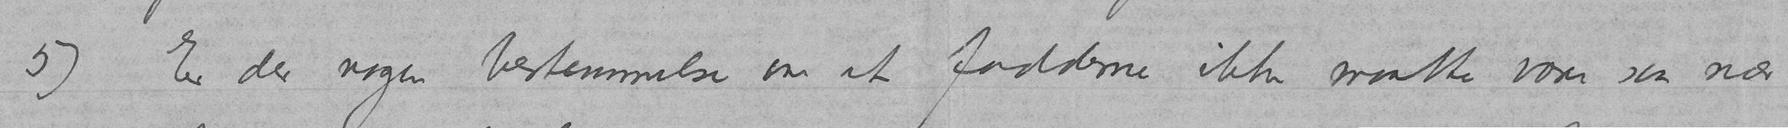5) Er der nogen bestemmelse om at fadderne ikke maatte være saa nær
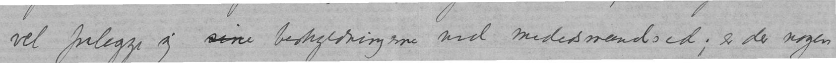vel fralegge sig sine beskyldningerne ved mededsmandsed; er der nogen
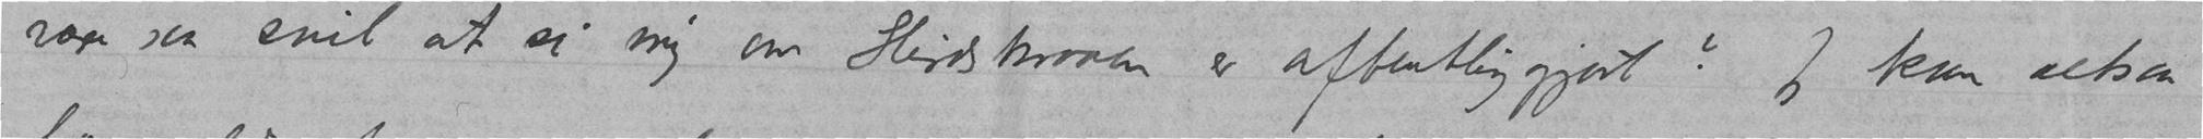være saa snil at si mig om Hirdskraaen er offentliggjort? Jeg kan altsaa
